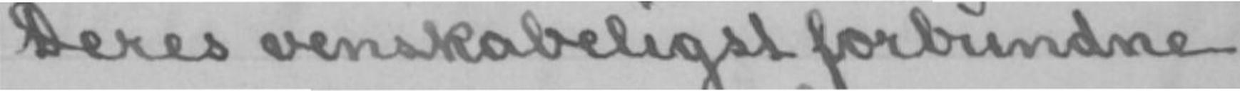Deres venskabeligst forbundne
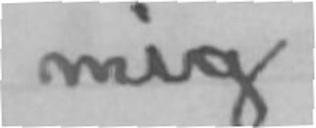mig
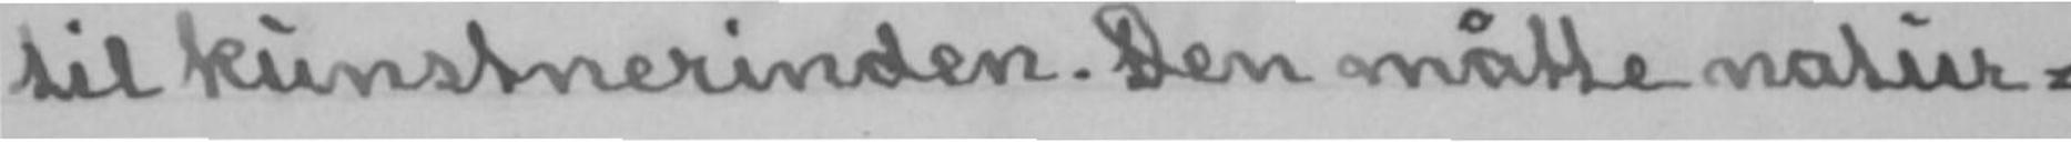til kunstnerinden. Den måtte natur-
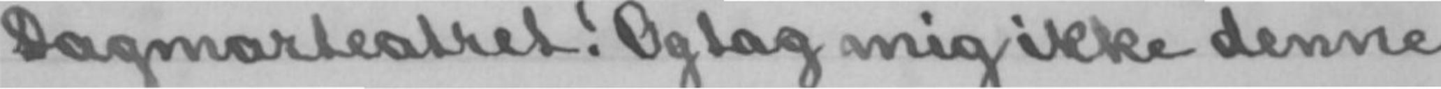Dagmarteatret! Og tag mig ikke denne
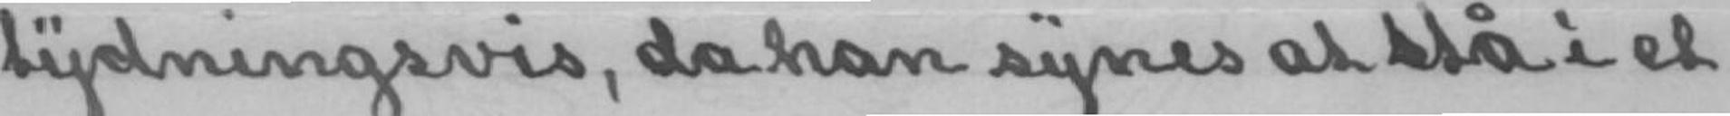tydningsvis, da han synes at stå i et
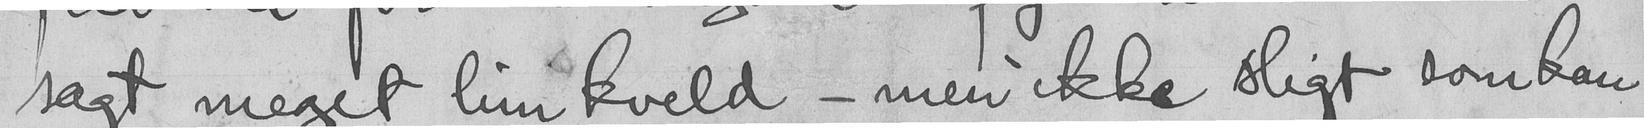sagt meget hin kveld - men ikke sligt som kan
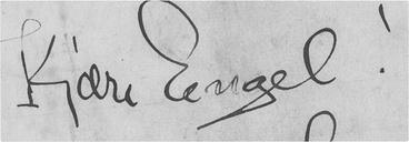Kjære Engel!
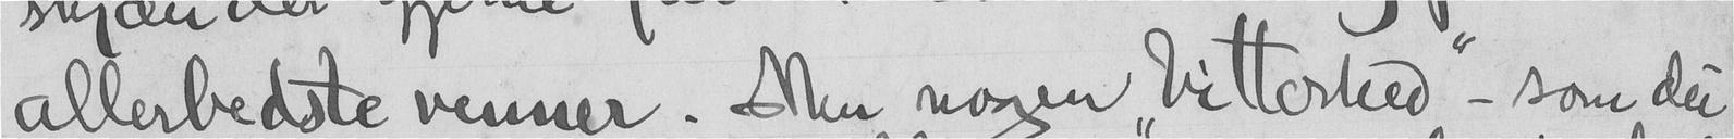allerbedste venner. Men nogen "bitterhed" - som du
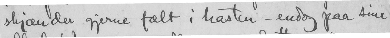skjænder gjerne fælt i hasten - endog paa sine
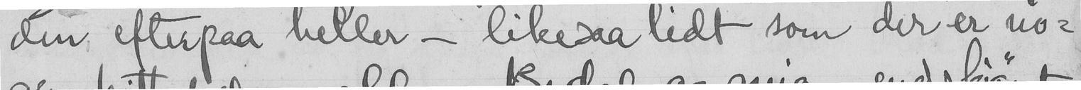den efterpaa heller - likesaa lidt som der er no-
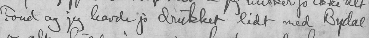Fond og jeg havde jo drukket lidt med Bydal
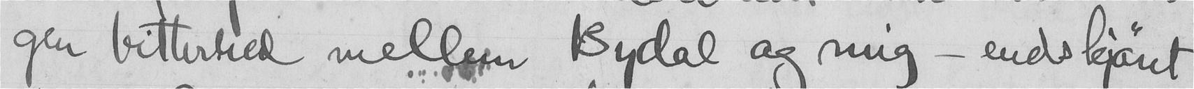gen bitterhed mellem Bydal og mig - endskjønet
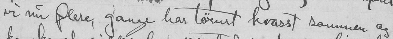vi nu flere gange har tørnet kvasst sammen og
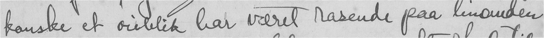kanske et øieblik har været rasende paa hinanden
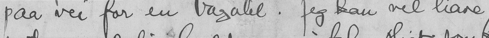paa vei for en bagatel. Jeg kan vel have
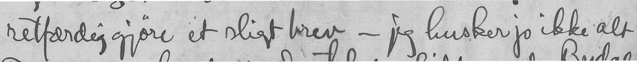retfærdiggjøre et sligt brev - jeg husker jo ikke alt
